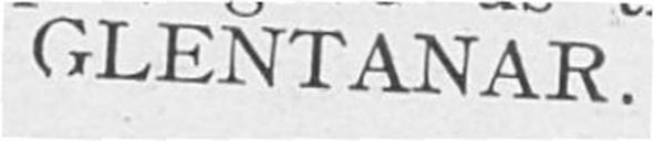GLENTANAR.
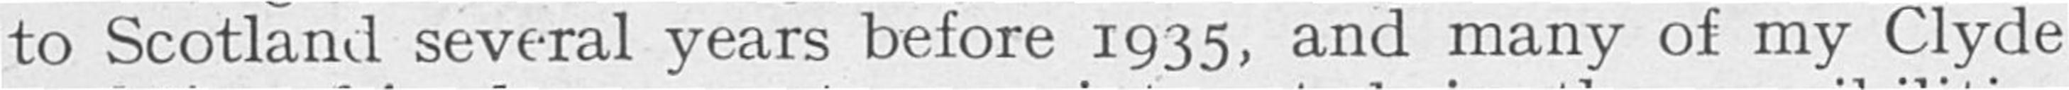to Scotland several years before 1935, and many of my Clyde
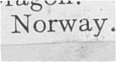Norway.
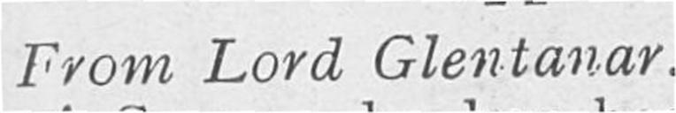From Lord Glentanar.
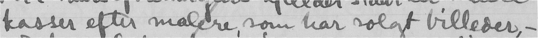kasser efter malere som har solgt billeder,-
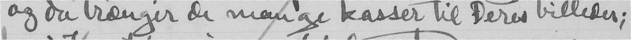og da trænger de mange kasser til Deres billeder;
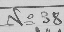No 38
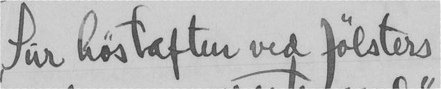"Sur høstaften ved Jølsters
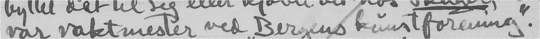var vaktmester ved "Bergens kunstforening".
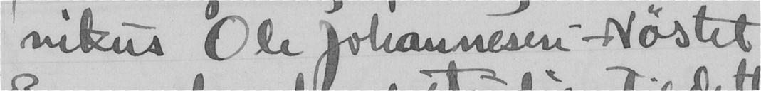nikus Ole Johannesen - Nøstet
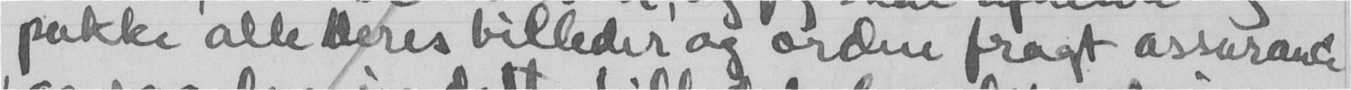pakke alle Deres billeder og ordne fragt assurand
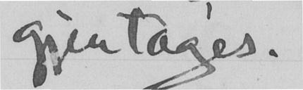gjentages.
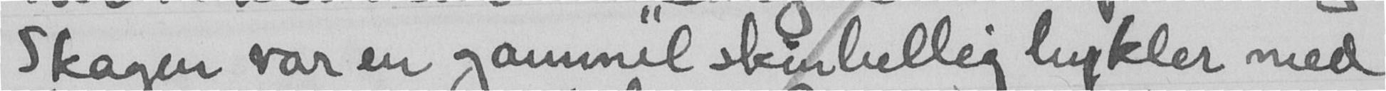Skagen var en gammel skinhellig hykler med
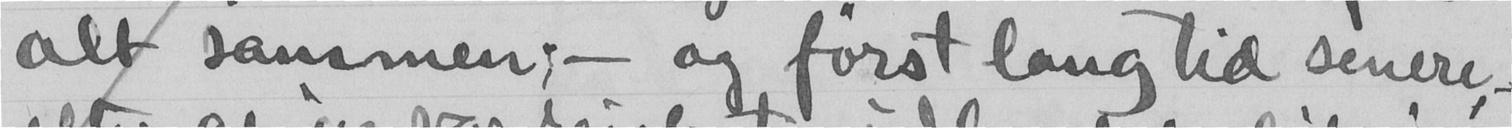alt sammen; - og først lang tid senere, -
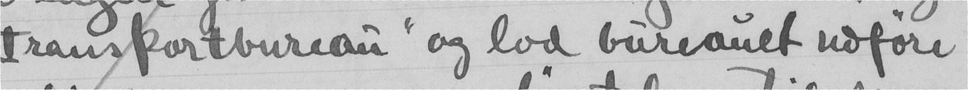Transportbureau" og lod bureauet udføre
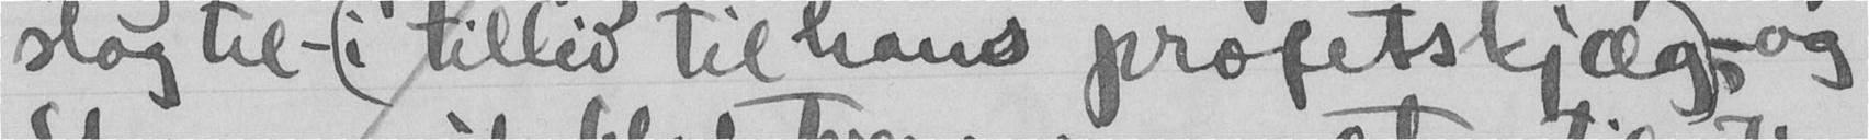slog til (i tillid til hans profetskjæg)- og
